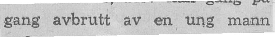gang avbrutt av en ung mann
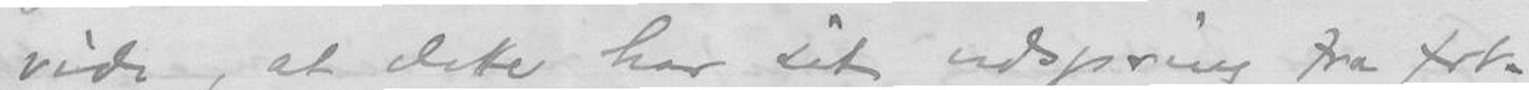vide, at dette har sit udspring fra frk.
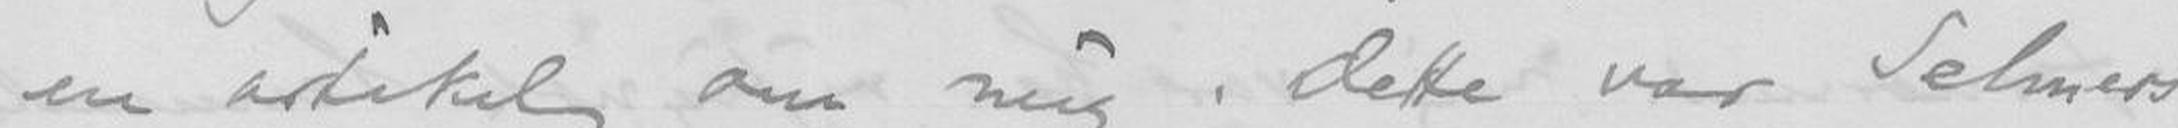en artikel om mig. Dette var Selmers
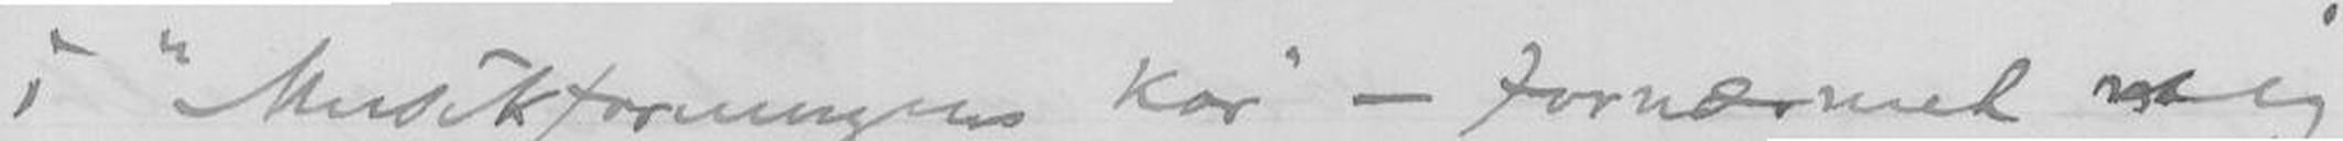i "Musikforeningens Kor" - fornærmed mig
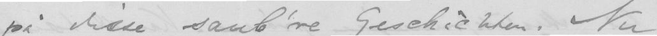på disse saub're Geschichten. Nu
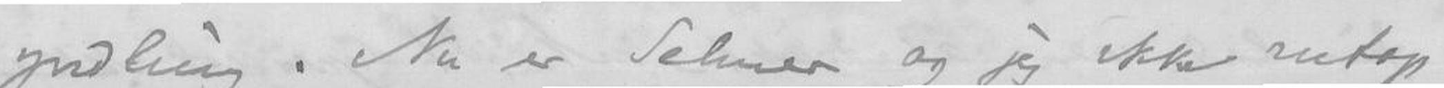yngling. Nu er Selmer og jeg ikke netop
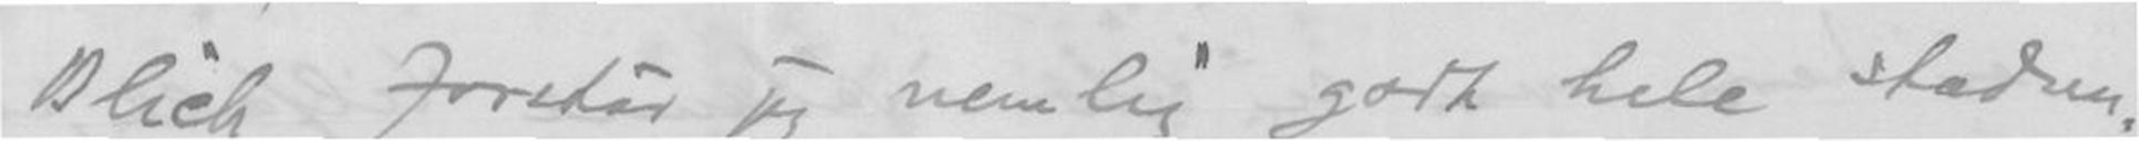Blich forstår jeg nemlig godt hele stadsen.
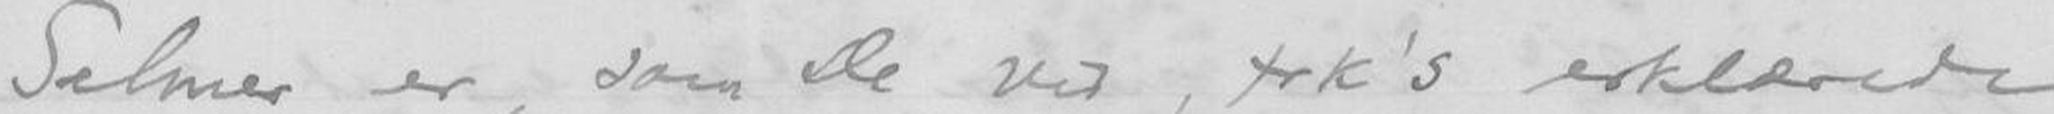Selmer er, som De ved, frk's erklærede
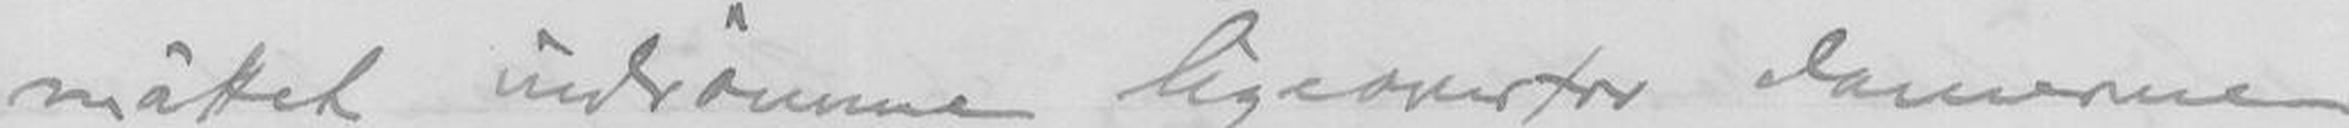måttet indrømme ligeoverfor damerne
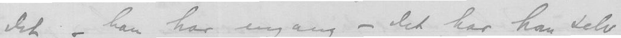det - han har engang - det har han selv
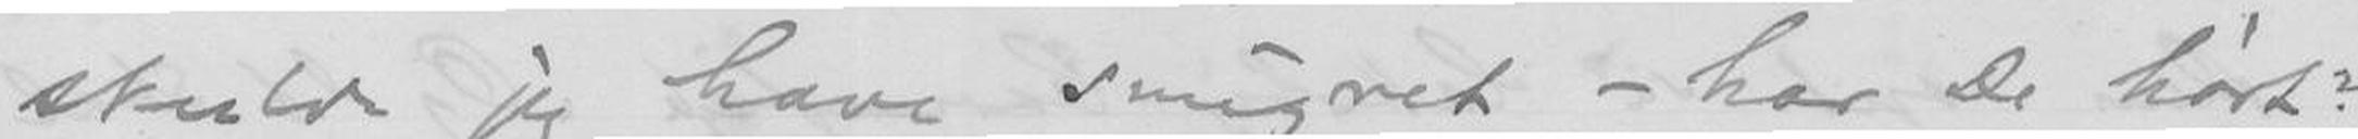skulde jeg have smigret - har De hørt?
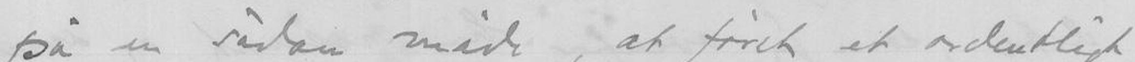på en sådan måde, at først et ordentligt
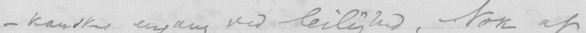- kanske engang ved leilighed. Nok af
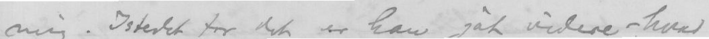mig. Istedet for det er han gåt videre - hvad
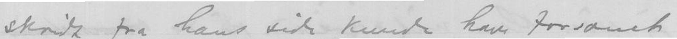skridt fra hans side kunde have forsomet
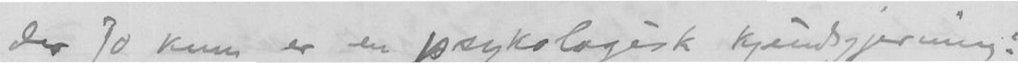der jo kun er en psykologisk kjendsgjerning!
